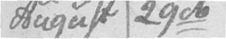August 29de
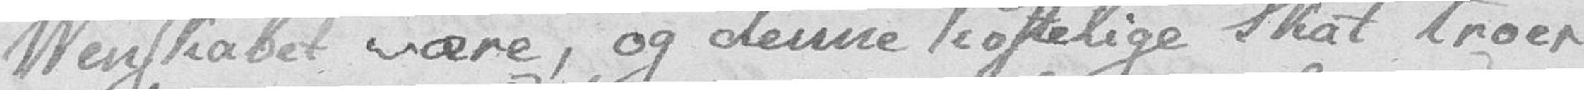Venskabet være, og denne kostelige Skat troer
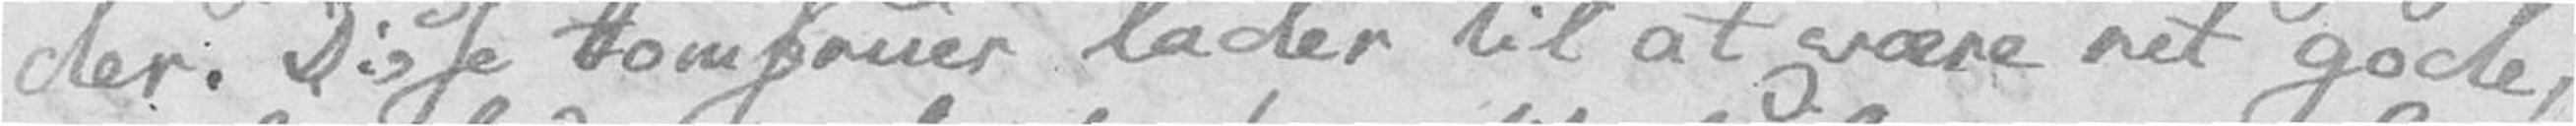der. Disse Jomfruer lader til at være ret gode,
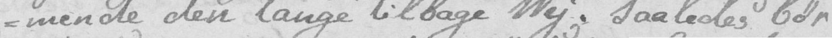-mende den lange tilbage Vej. Saaledes bør
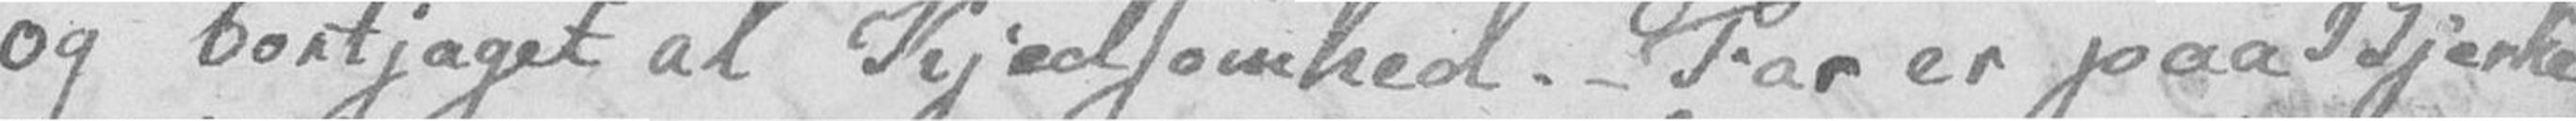og bortjaget al Kjedsomhed. Far er paa Bjerke
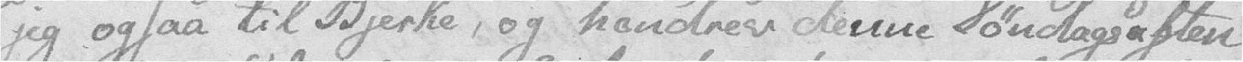jeg ogsaa til Bjerke, og hendrev denne Søndagsaften
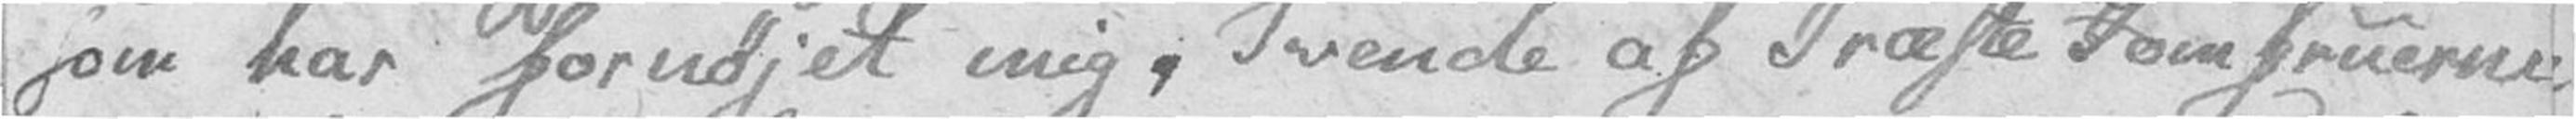som har fornøjet mig, Tvende af Præste Jomfruerne
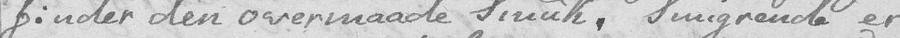finder den overmaade Smuk. Smigrende er
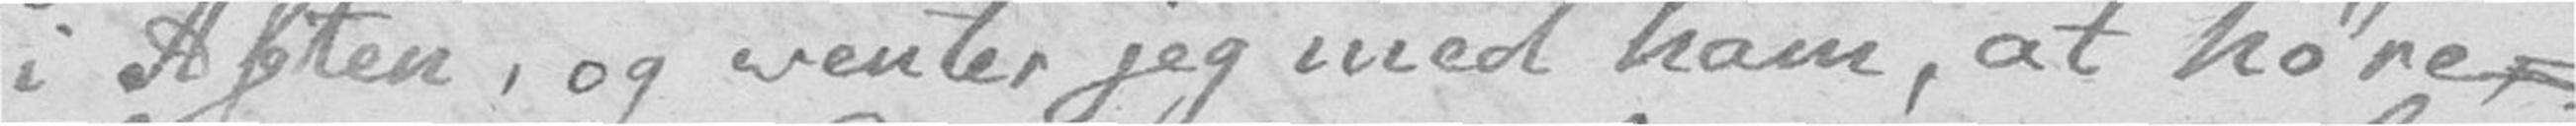i Aften, og venter jeg med ham, at høre,
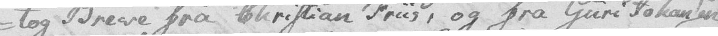-tog Breve fra Christian Friis, og fra Guri Johansen
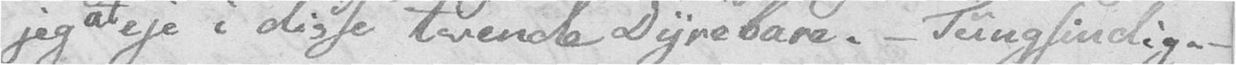jeg at eje i disse tvende Dyrebare. – Tungsindig. –
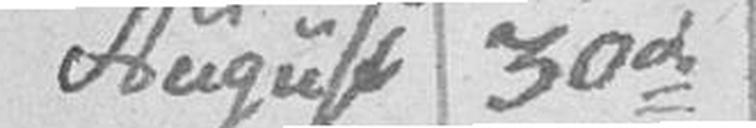August 30d
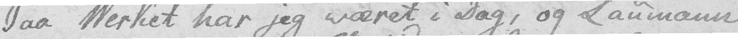Paa Verket har jeg været i Dag, og Laumann
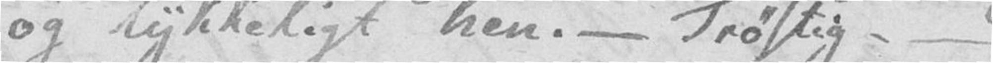og lykkeligt hen. – Trøstig. –
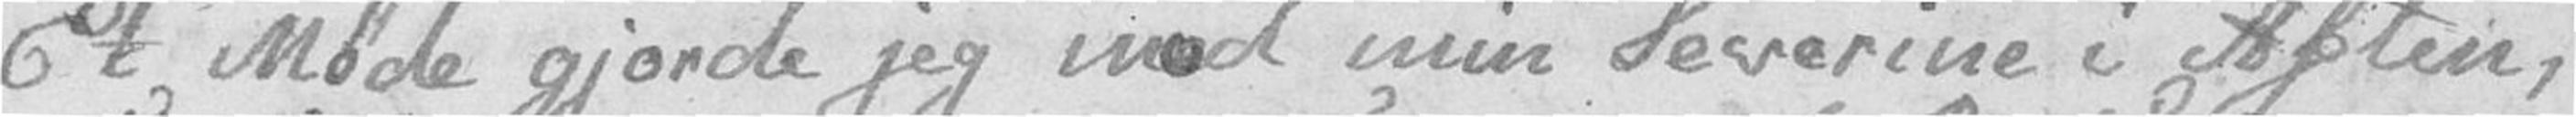Et Møde gjorde jeg med min Severine i Aften,
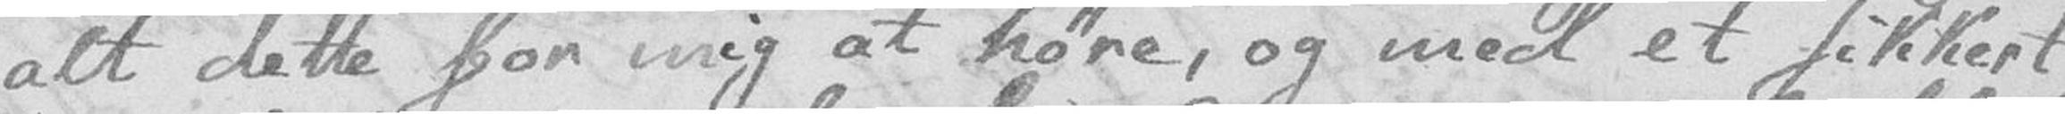alt dette for mig at høre, og med et sikkert
